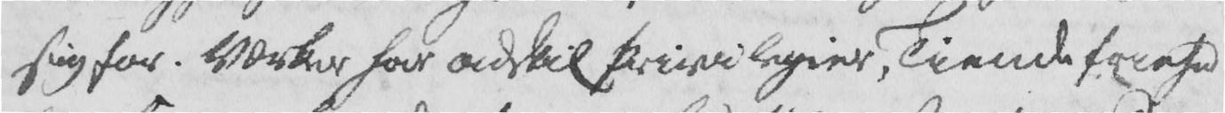sig for. Værker har adskil Privilegier, Tiende friehed
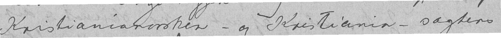Kristianianorsken - og Kristiania - sagtens
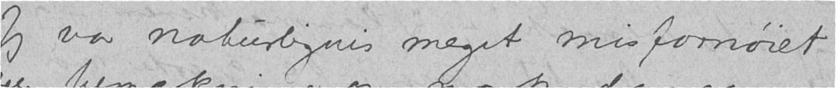Jeg var naturligvis meget misfornøiet
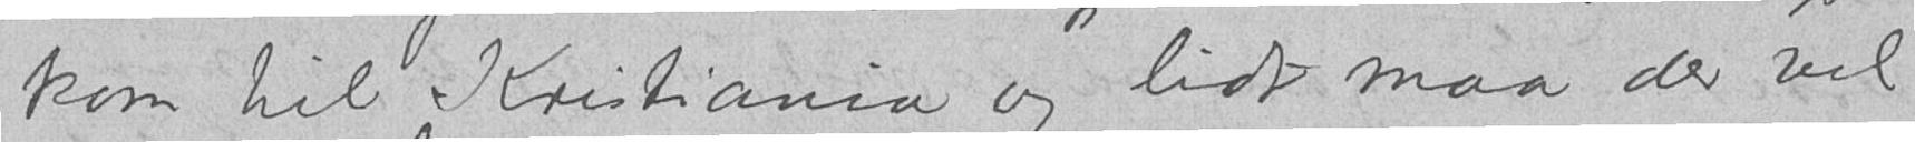kom til Kristiania og lidt maa der vel
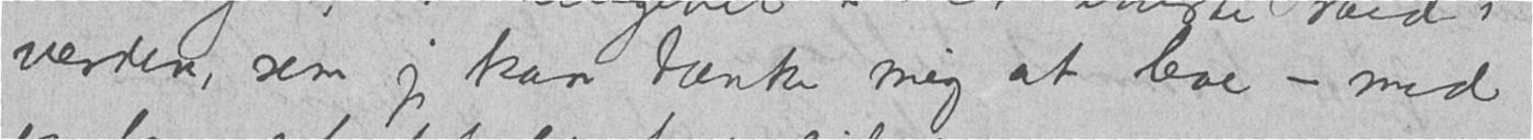verden, som jeg kan tænke mig at leve - med
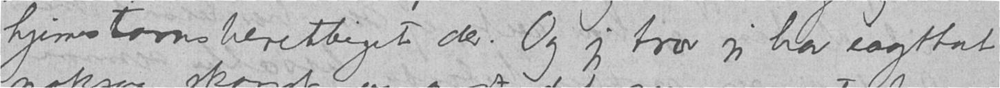hjemstavnsberettiget der. Og jeg tror jeg har iagttat
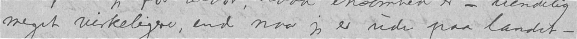meget virkeligere end naar jeg er ude paa landet -
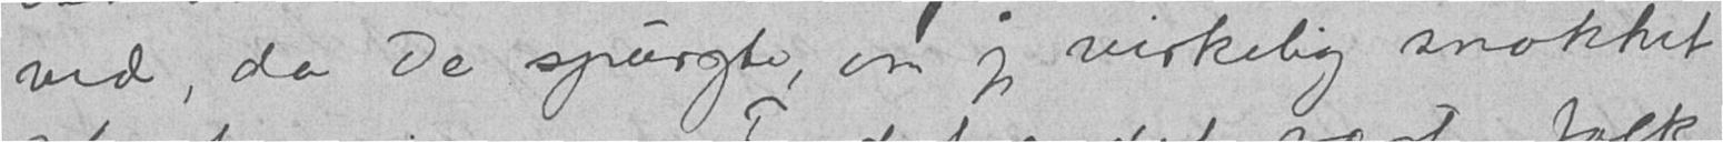ved da De spurgte, om jeg virkelig snakket
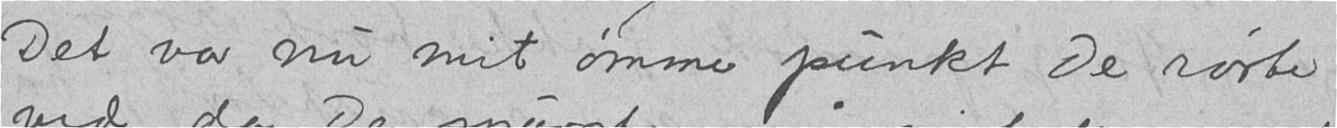Det var nu mit ømme punkt De rørte
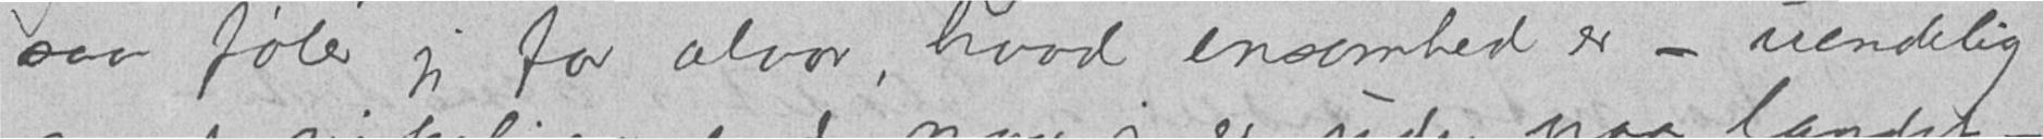saa føler jeg for alvor, hvad ensomhed er - uendelig
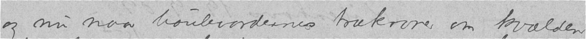og nu naar boulevardernes trækroner om kvælden
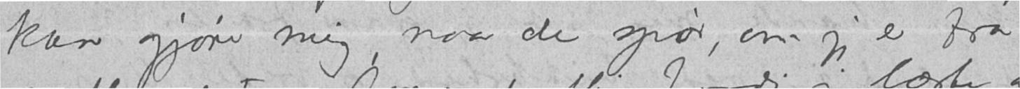kan gjøre mig, naar de spør, om jeg er fra
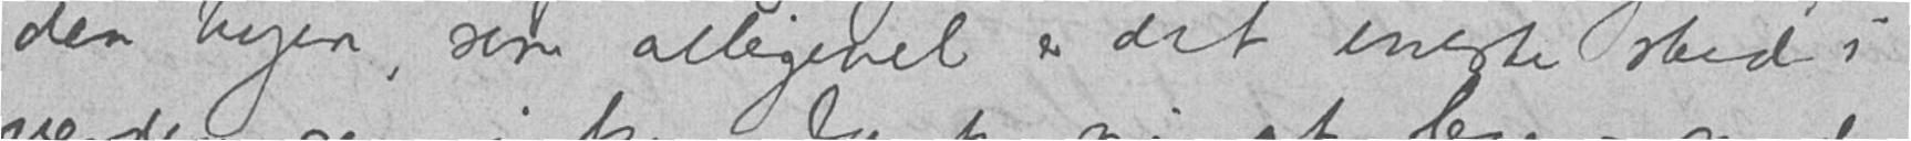den byen, som alligevel er det eneste sted i
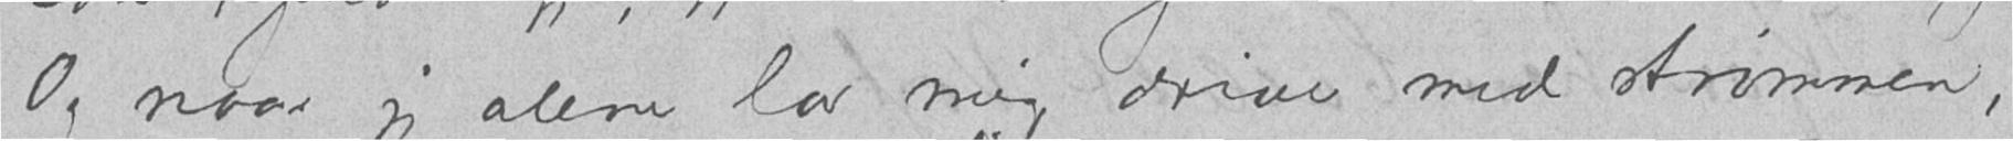Og naar jeg alene lar mig drive med strømmen,
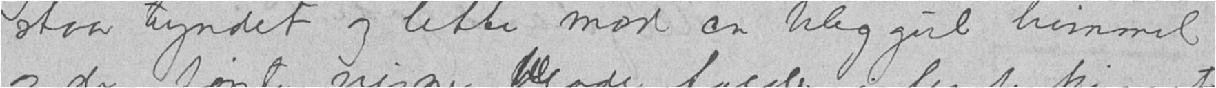staar tyndet og lette mod en bleggul himmel
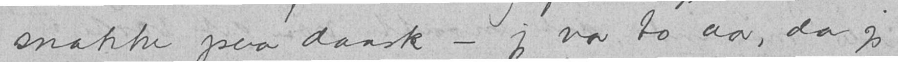snakke paa dansk - jeg var to aar, da jeg
